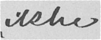ikke
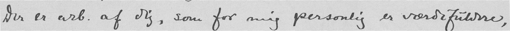Der er arb. af dig, som for mig personlig er værdifuldere,
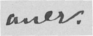aner.
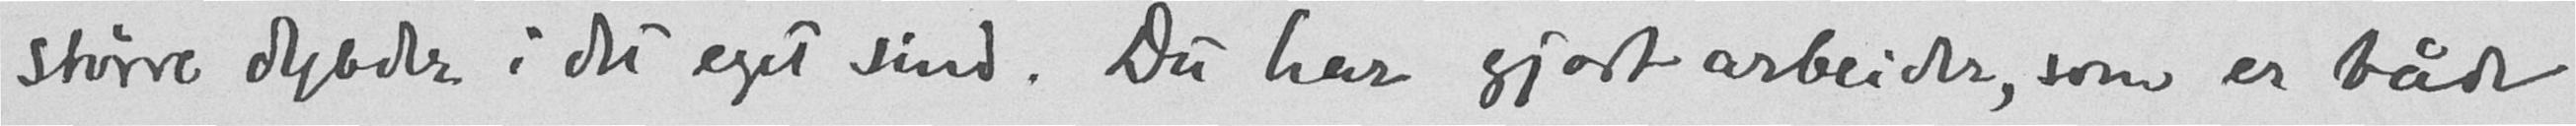større dybder i det eget sind. Du har gjort arbeider, som er både
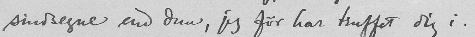sindsegne end dem, jeg før har truffet dig i.
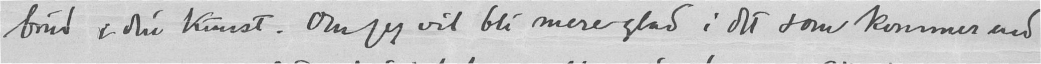brud i din kunst. Om jeg vil bli mere glad i det som kommer end
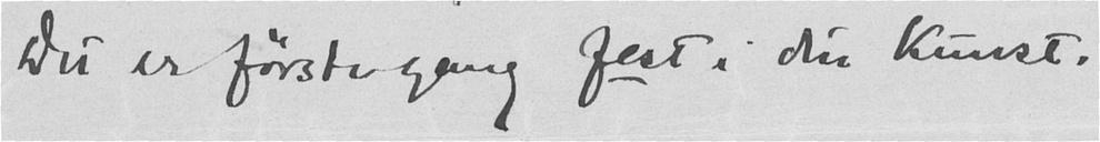Det er første gang fest i din kunst.
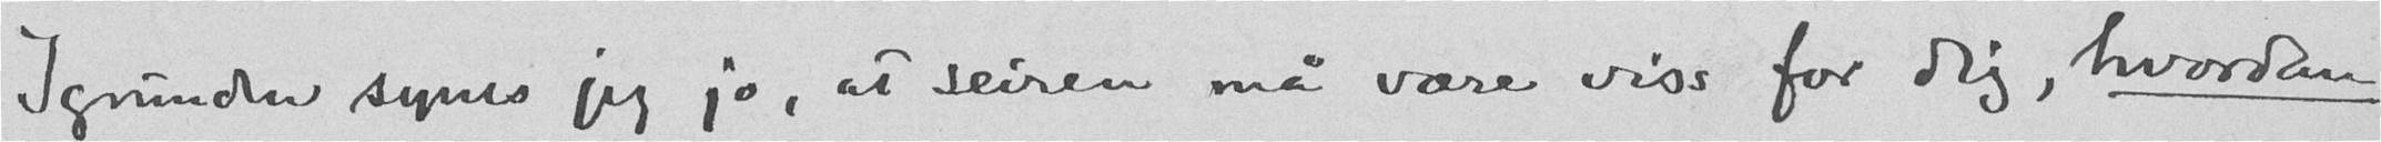I grunden synes jeg jo, at seiren må være viss for dig, hvordan
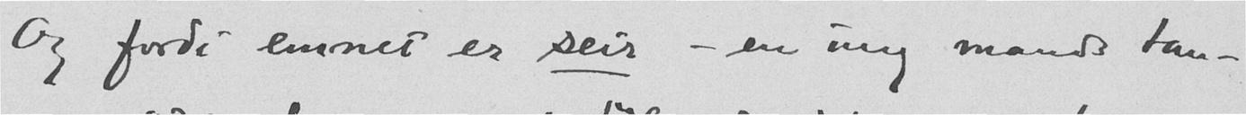Og fordi emnet er seir - en ung mands tan-
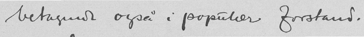betagende også i populær forstand.
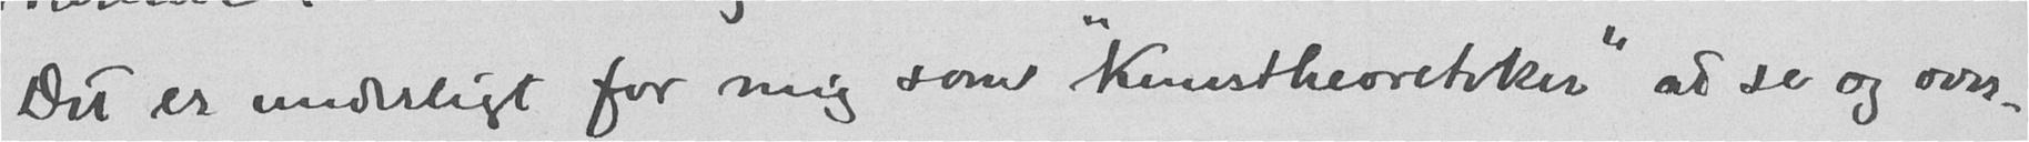Det er underligt for mig som "kunstheoretiker" at se og over-
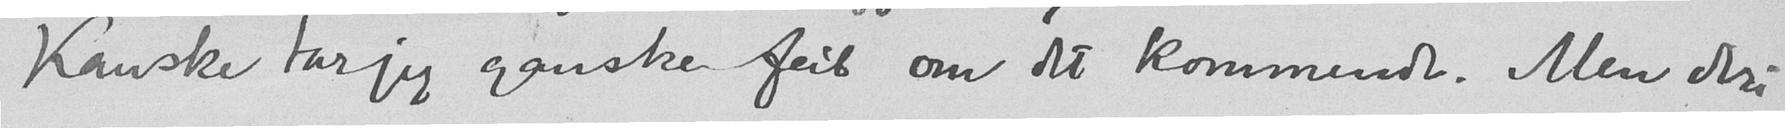Kanske tar jeg ganske feil om det kommende. Men deri
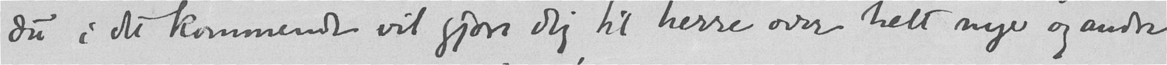du i det kommende vil gjøre dig til herre over helt nye og andre
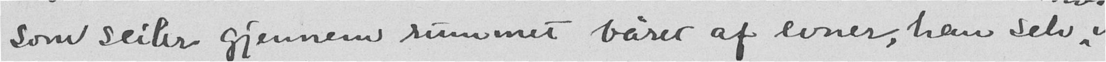som seiler gjennem rummet båret af evner, han selv,
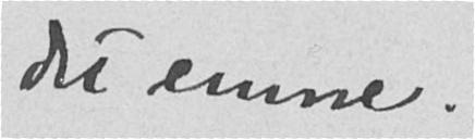det emne.
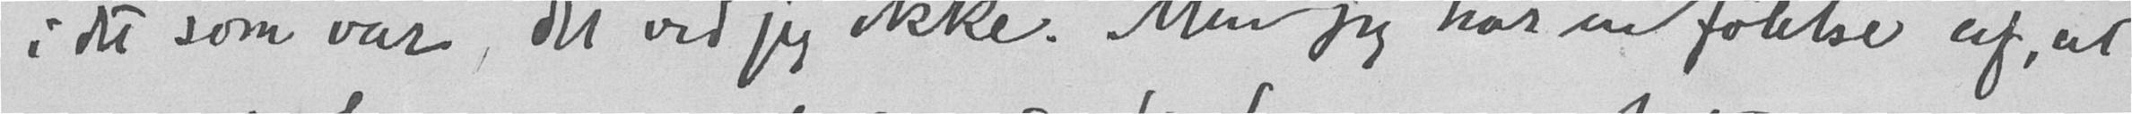i det som var, det ved jeg ikke. Men jeg har en følelse af, at
